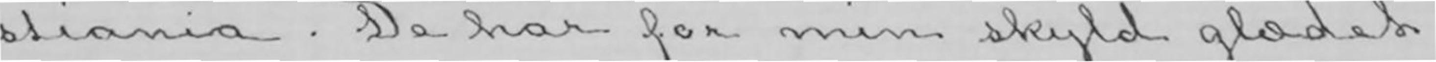stiania. De har for min skyld glædet
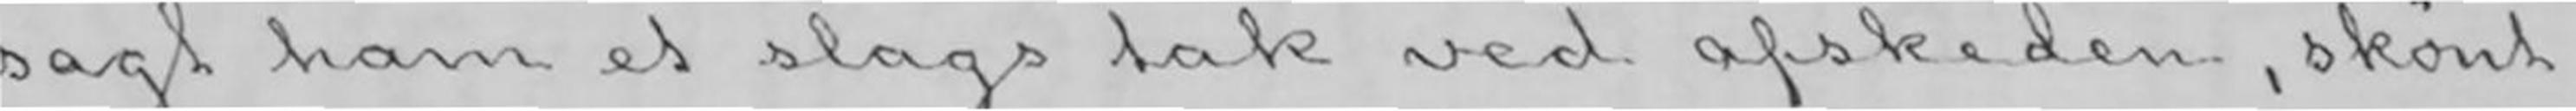sagt ham et slags tak ved afskeden, skønt
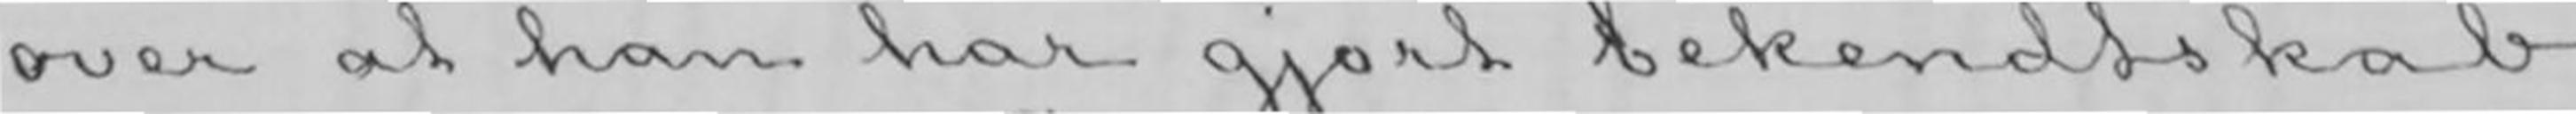over at han har gjort bekendtskab
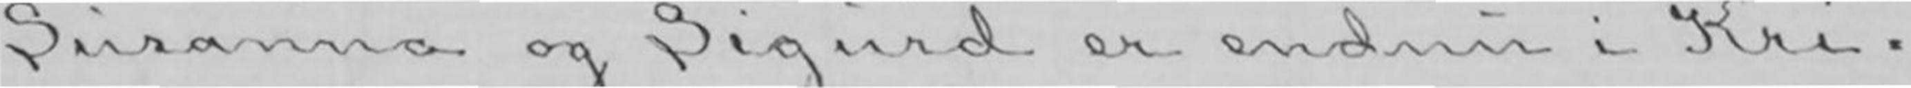Susanna og Sigurd er endnu i Kri-
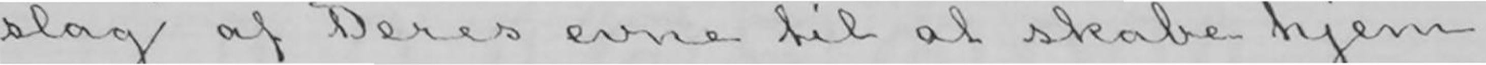slag af Deres evne til at skabe hjem
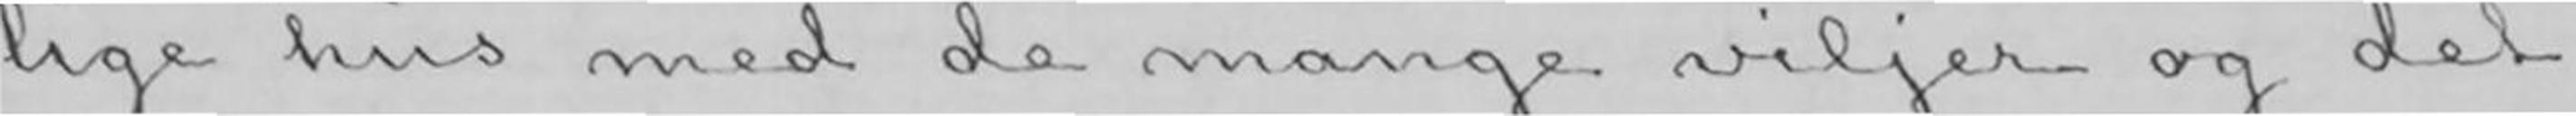lige hus med de mange viljer og det
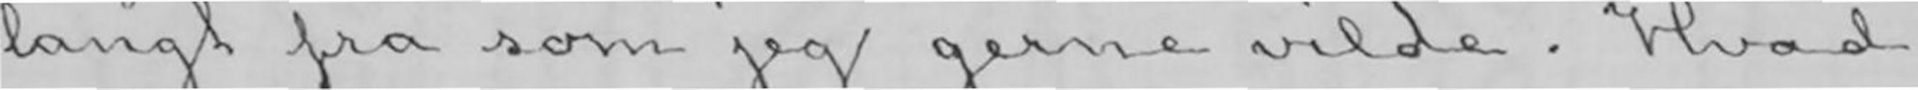langt fra som jeg gerne vilde. Hvad
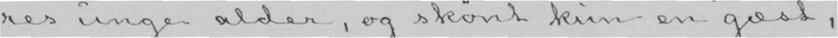res unge alder, og skønt kun en gæst,
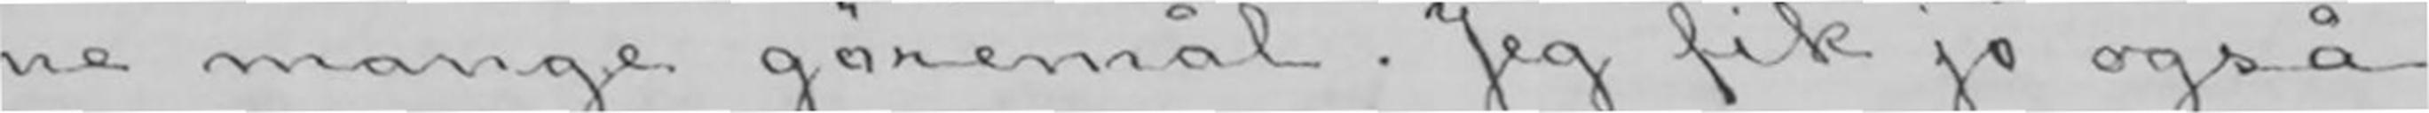ne mange gøremål. Jeg fik jo også
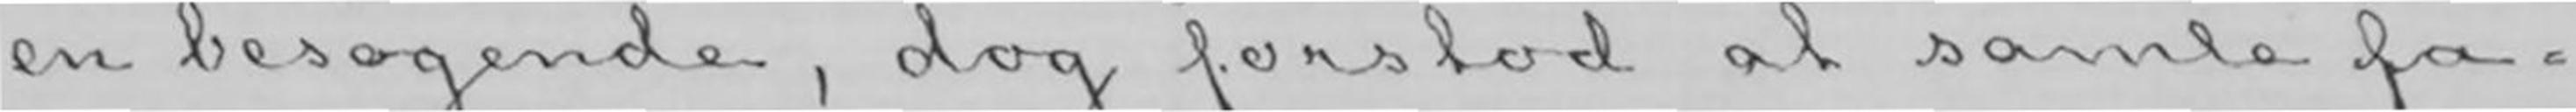en besøgende, dog forstod at samle fa-
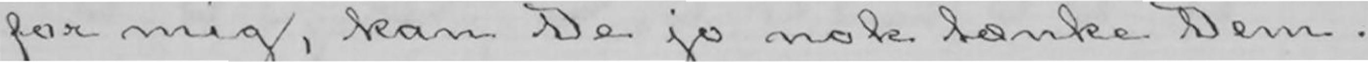for mig, kan De jo nok tænke Dem.-
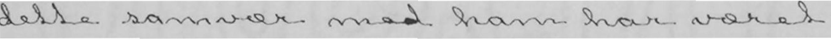dette samvær med ham har været
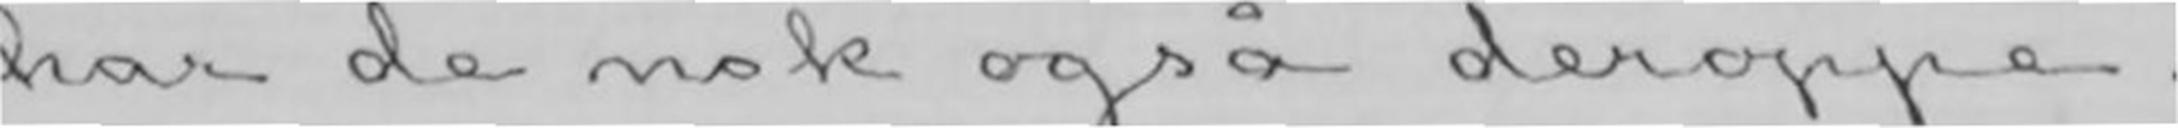har de nok også deroppe.-
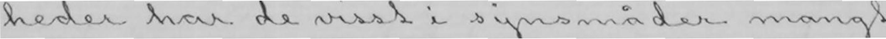heder har de visst i synsmåder mangt
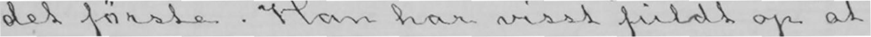det første. Han har visst fuldt op at
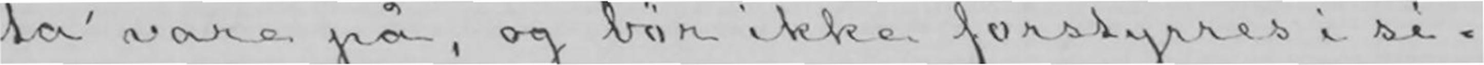ta´ vare på, og bør ikke forstyrres i si-
